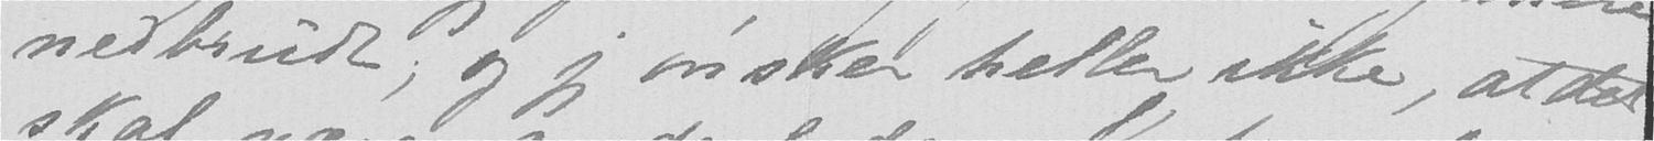nedbrudt, og jeg ønsker heller ikke, at det
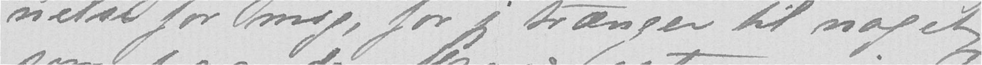nelse for mig, for jeg trænger til noget
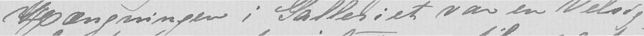Hængningen i Galleriet var en Velsig-
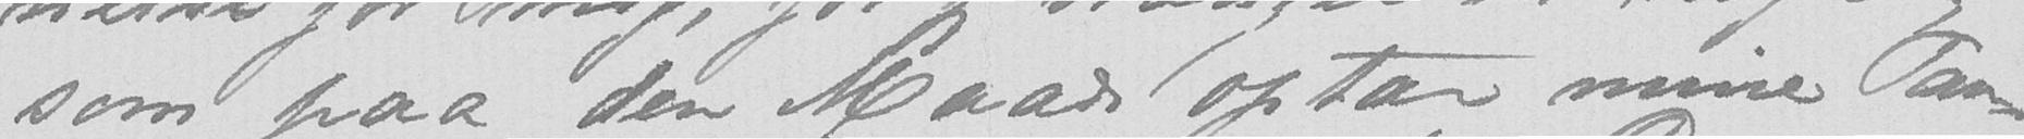som paa den Maade optar mine Tan-
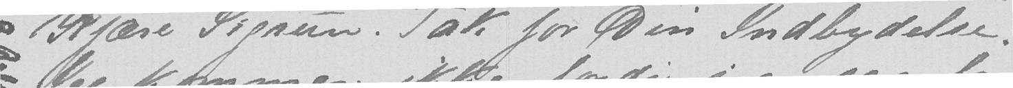Kjære Sigrun. Tak for Din Indbydelse.
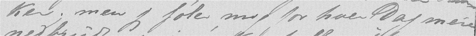ker; men jeg føler mig for hver Dag mere
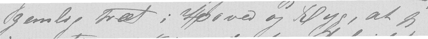gemlig træt i Hoved og Ryg, at jeg
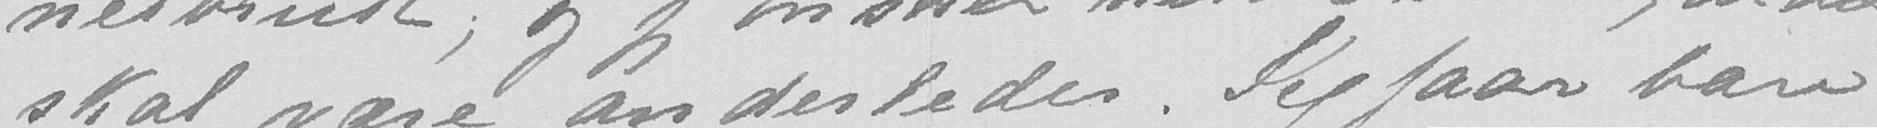skal være anderledes. Jeg faar bare
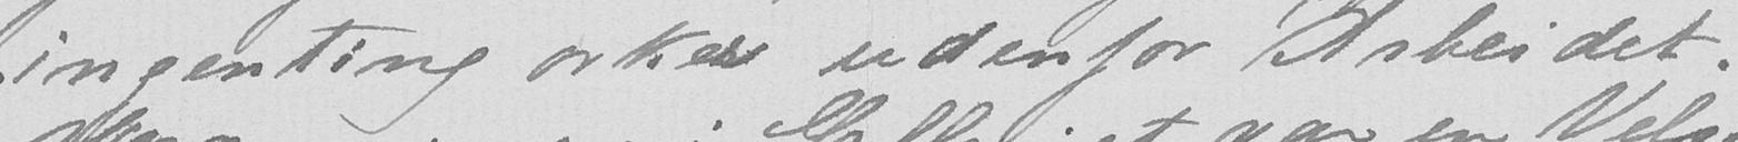ingenting orker udenfor Arbeidet.
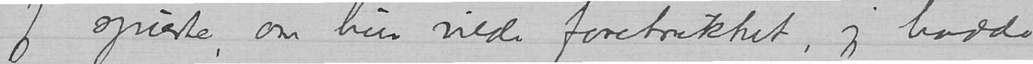Jeg spurte, om hun vilde foretrukket, jeg hadde
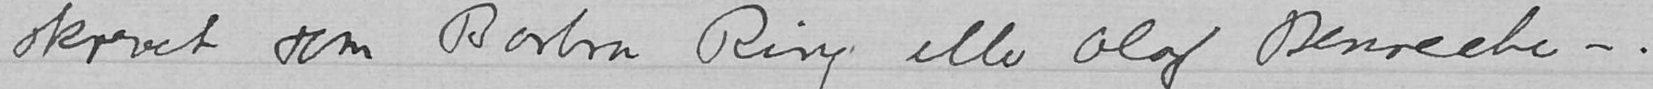skrevet som Barbra Ring eller Olaf Benneche-.
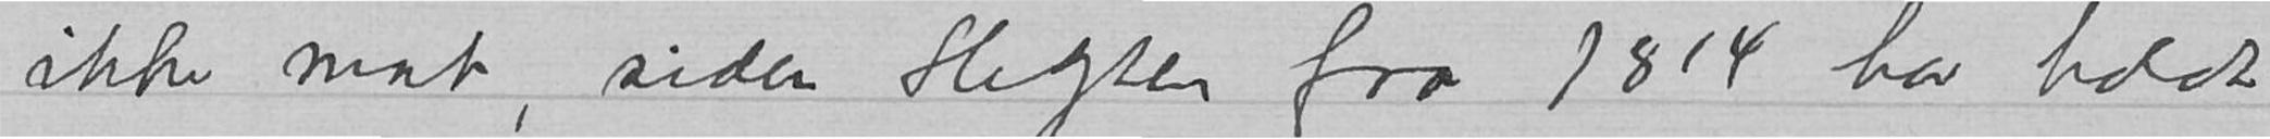ikke mat, siden slægten fra 1814 har holdt
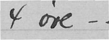4 øre -.
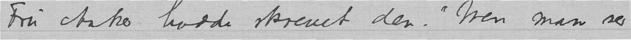Fru Anker hadde skrevet den. «Men man ser
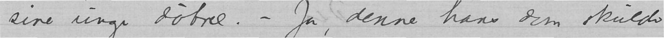sine unge døtre. – Ja, denne hans dom skulde
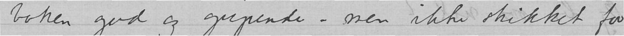boken god og gripende – men ikke skikket for
